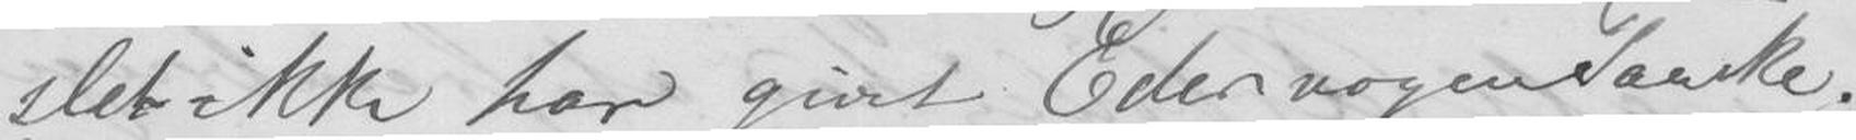slet ikke har givet Eder nogen Tanke.
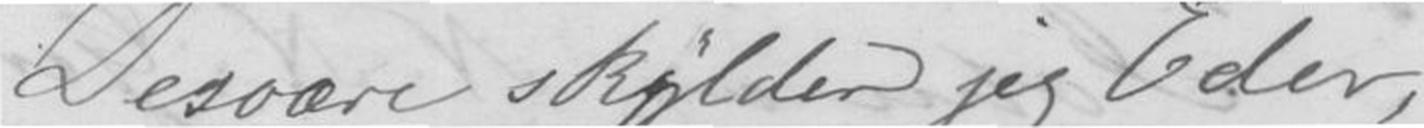Desvære skylder jeg Eder,
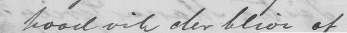hvad vil der blive af
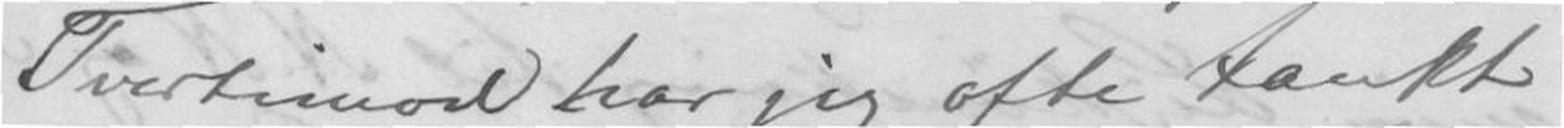Tvertimod har jeg ofte tænkt
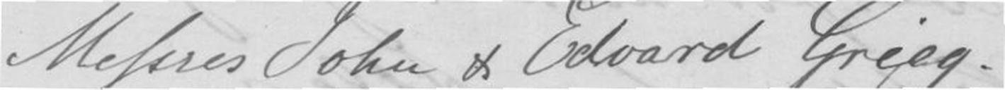Messres John & Edvard Grieg.
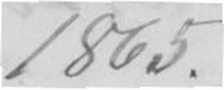1865.
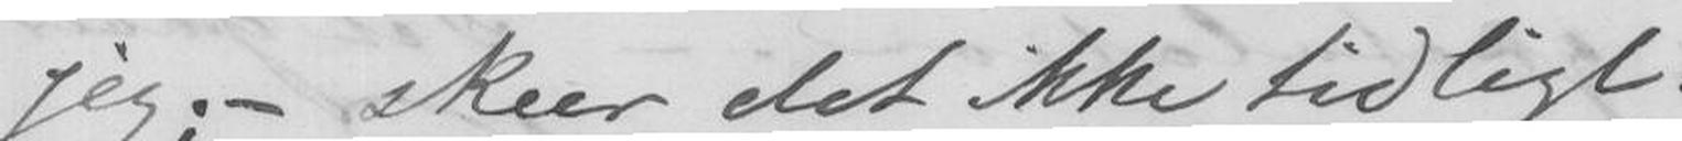jeg; - skeer det ikke tidligt
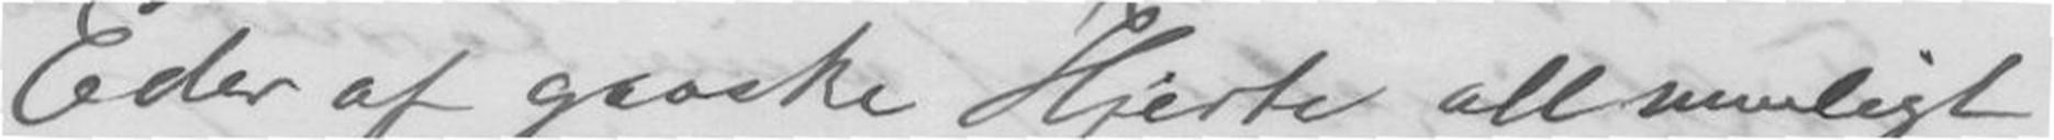Eder af ganske Hjerte allmuligt
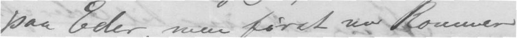paa Eder, men først nu kommer
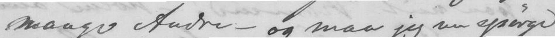mange Andre - og maa jeg nu spørge
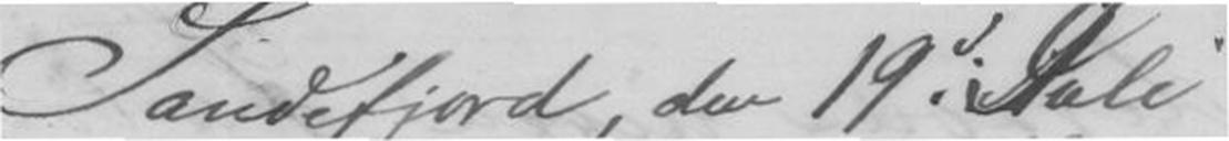Sandefjord, den 19d Juli
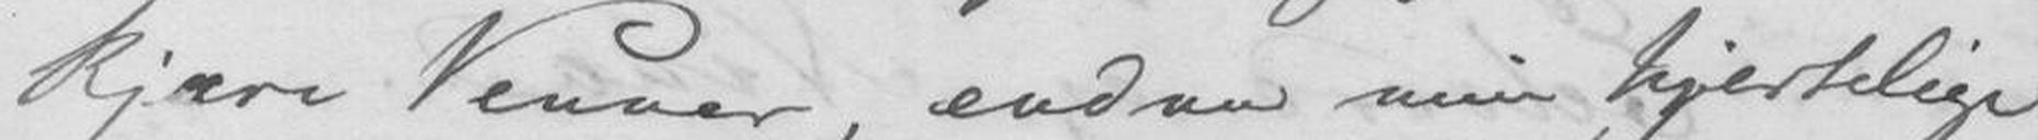kjære Venner, endnu min hjertelige
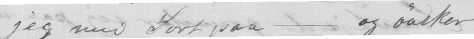jeg med Sort paa - og ønsker
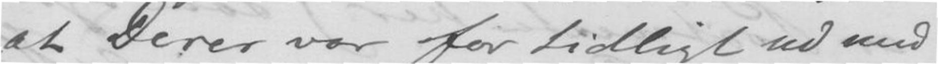at Derer var got tidligt ud med
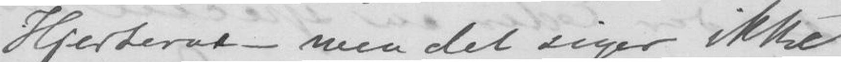Hjerterne - men det siger ikke
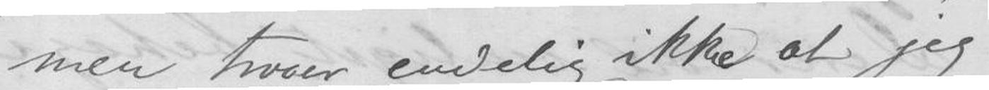men trooer endelig ikke at jeg
